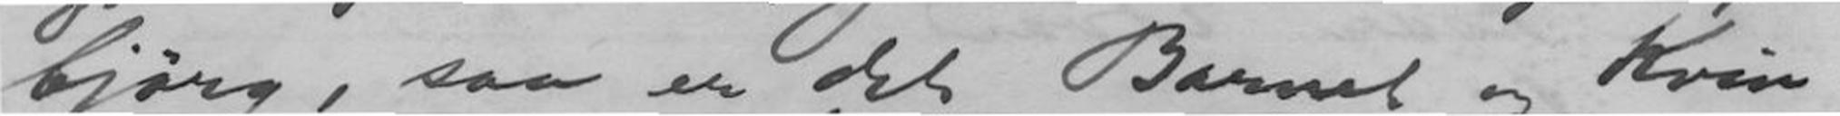bjørg, saa er det Barnet og Kvin-
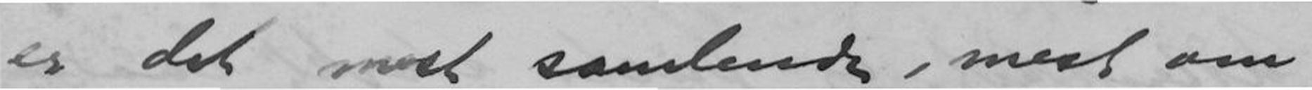er det mest samlende, mest om-
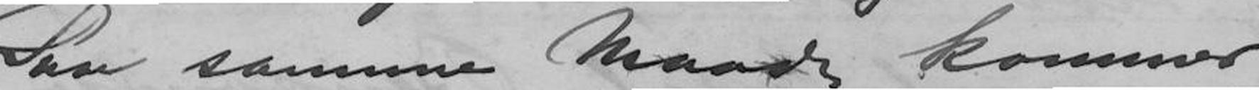Paa samme Maade kommer
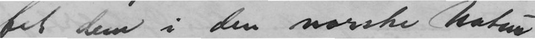fet dem i den norske Natur,
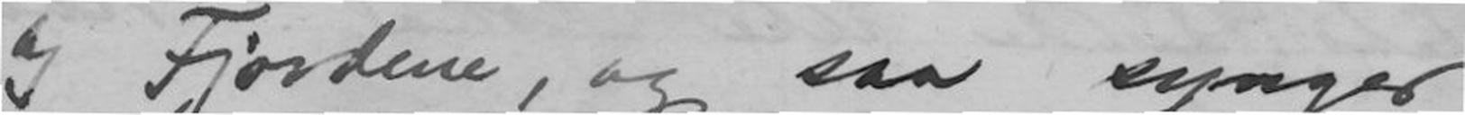og Fjordene, og saa synger
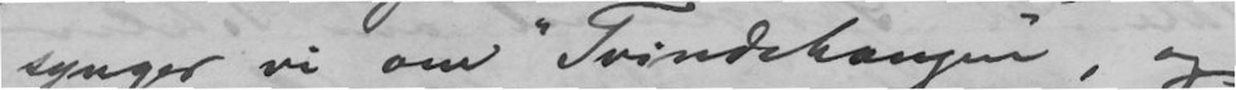synger vi om "Tvindehougen", og
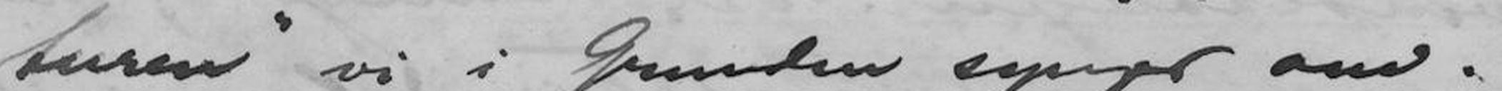turen" vi i Grunden synger om.
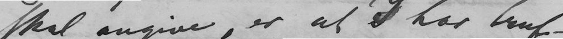skal angive, er at I har truf-
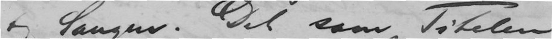og Sangen. Det som Titelen
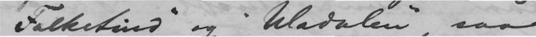"Falketind" og "Uladalen", saa
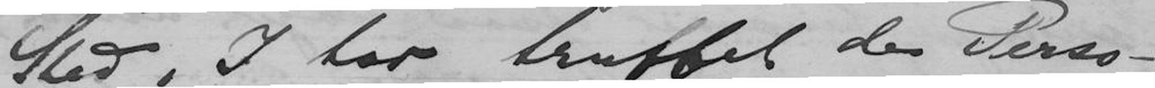Sted, I har truffet de Perso-
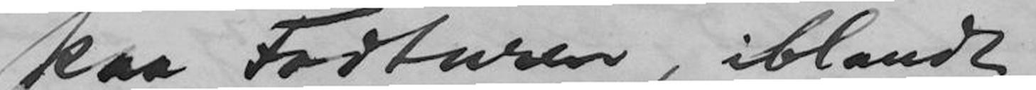paa Fodturen, iblandt
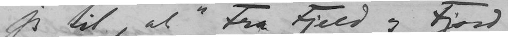jeg til, at "Fra Fjeld og Fjord"
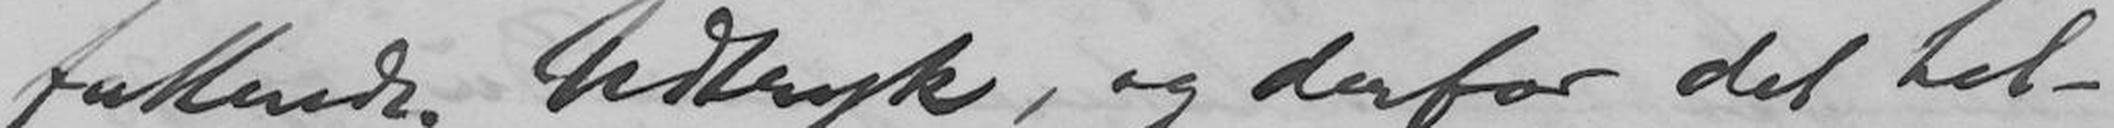fattende Udtryk, og derfor det hel-
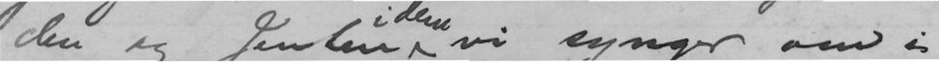den og Jenten vi synger om i
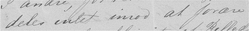deles intet imod at forære
